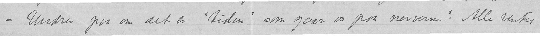– Undres paa om det er "tiden" som gaar os paa nerverne? Alle venter
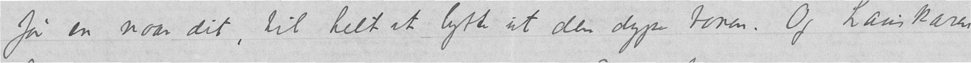før en naar dit, til helt at bytte ut den dype tonen. Og Lauskaren
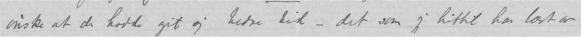ønske at de hadde git sig bedre tid – det som jeg hittil har læst av
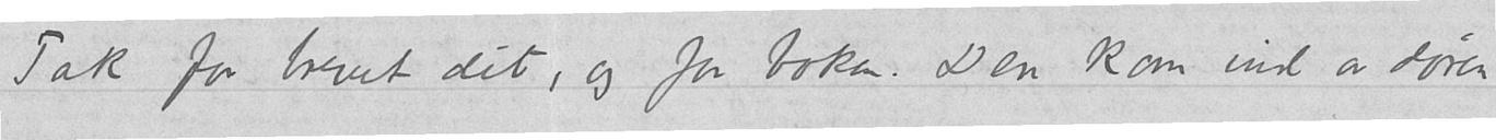Tak for brevet dit, og for boken. Den kom ind av døren
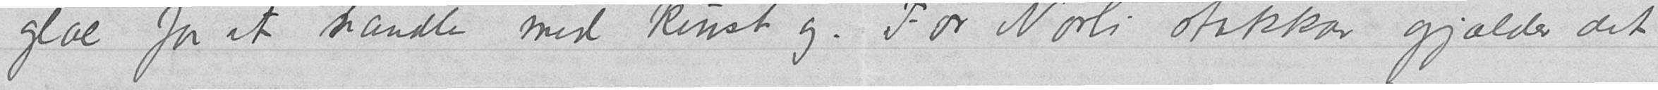glae for at handle med kunst og. For Norli stakkar gjælder det
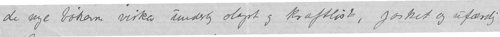de nye bøkerne virker underlig slapt og kraftløst, jasket og ufærdig
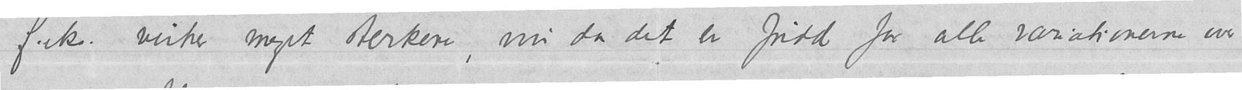f.eks. virker meget sterkere, nu da det er fridd for alle variationerne over
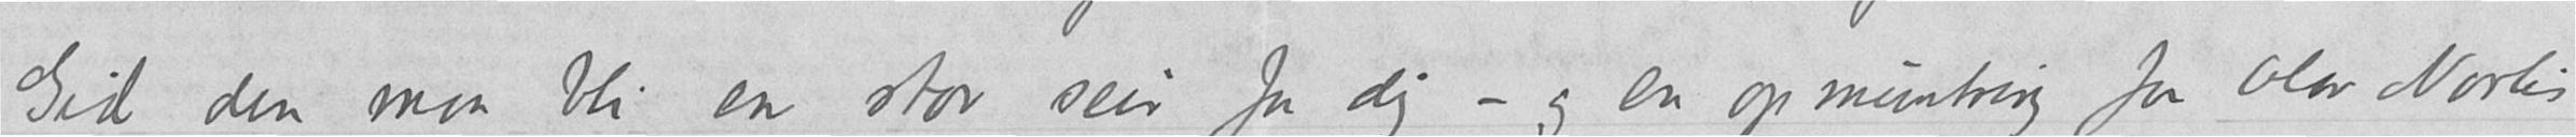Gid den maa bli en stor seir for dig – og en opmuntring for Olav Norlis
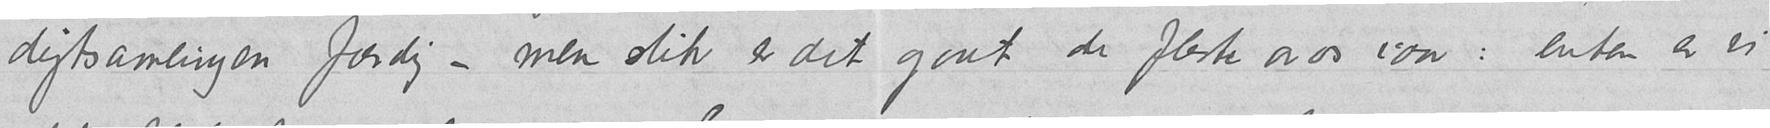digtsamlingen færdig – men slik er det gaat de fleste av os iaar: enten er vi
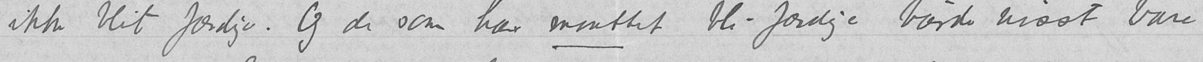ikke blit færdige. Og de som har maattet bli færdige burde visst bare
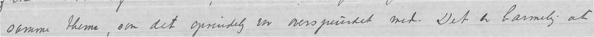samme thema, som det oprindelig var overspundet med. Det er harmelig at
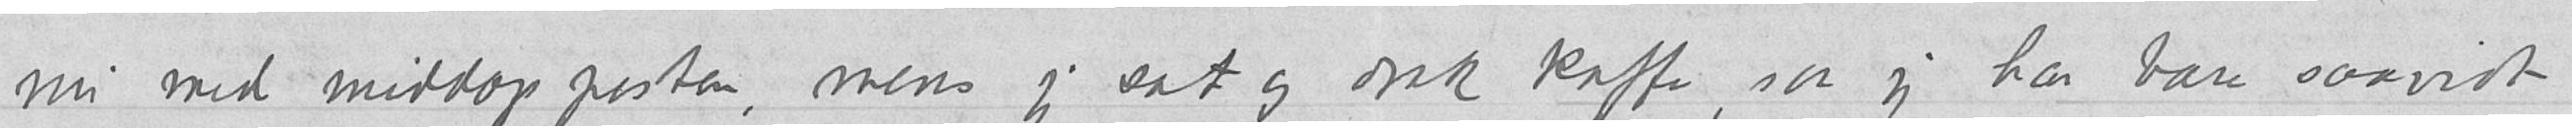nu med middagsposten, mens jeg sat og drak kaffe, saa jeg har bare saavidt
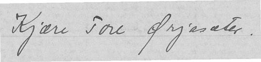Kjære Tore Ørjasæter.
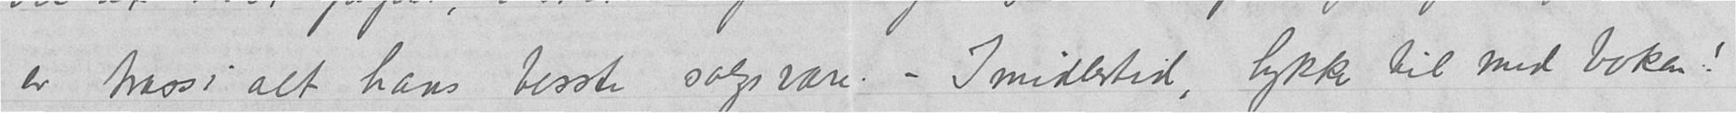er trass i alt hans besste solgsvare. – Imidlertid, lykke til med boken!
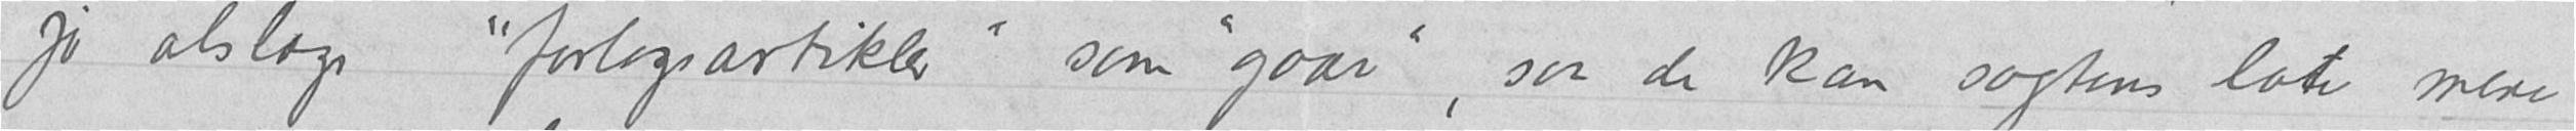jo alslags «forlagsartikler» som gaar, saa de kan sagtens late mere
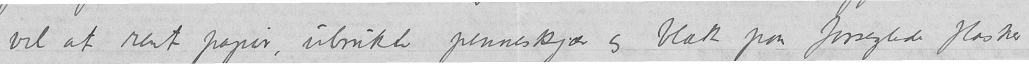vel at rent papir, ubrukte penneskjær og blæk paa forseglede flasker
